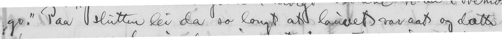go." Paa slutten lei da so longt at lauvet var aat og datte
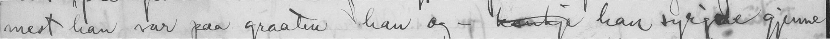mest han var paa graaten han og -  han syrjde gjenne
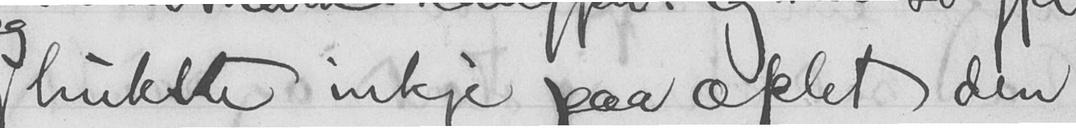hukste inkje paa æplet den
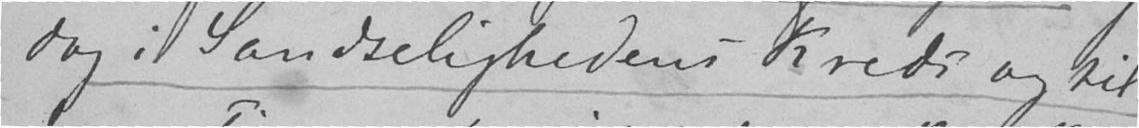dog i Sandselighedens Kreds og til
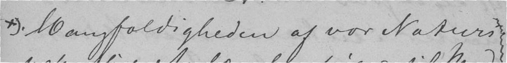Mangfoldigheden af vor Naturs
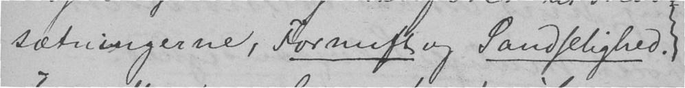sætningerne, Fornuft og Sandselighed.
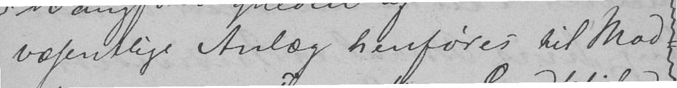væsentlige Anlæg henføres til Mod-
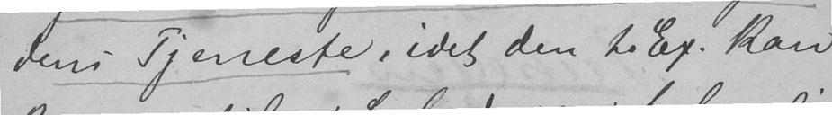dens Tjeneste, idet den t. Ex. kan
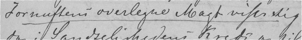Fornuftens overlegne Magt viser sig
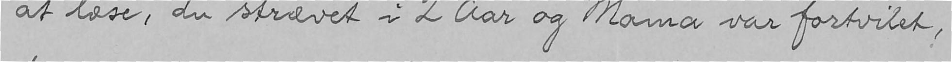at læse, du strævet i 2 Aar og Mama var fortvilet,
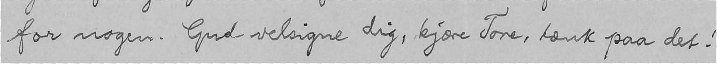for nogen. Gud velsigne dig, kjære Tore, tænk paa det!
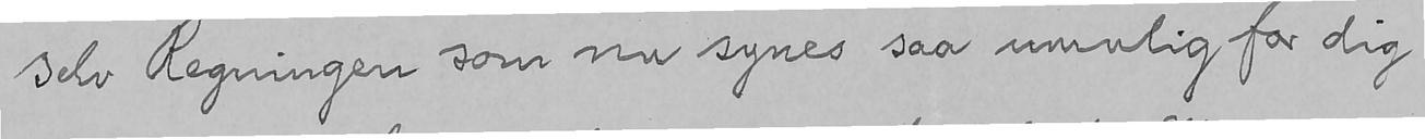selv Regningen som nu synes saa umulig for dig
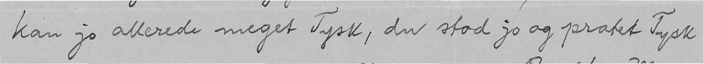kan jo allerede meget Tysk, du stod jo og pratet Tysk
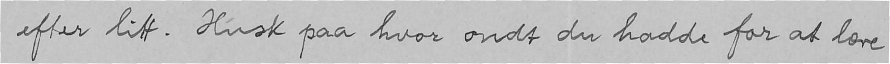efter litt. Husk paa hvor ondt du hadde for at lære
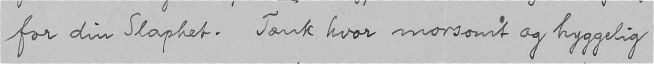for din Slaphet. Tænk hvor morsomt og hyggelig
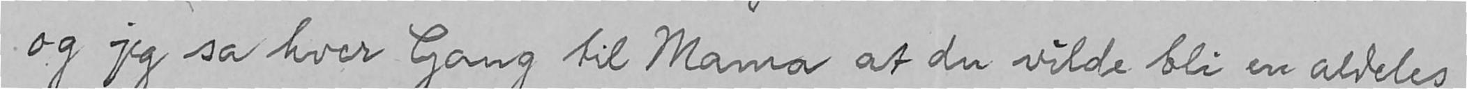og jeg sa hver Gang til Mama at du vilde bli en aldeles
